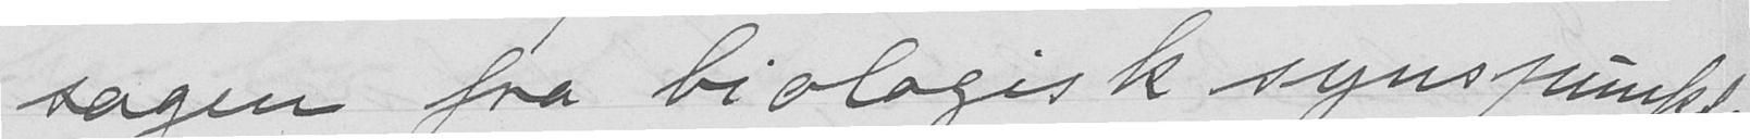sagen fra biologisk synspunkt",
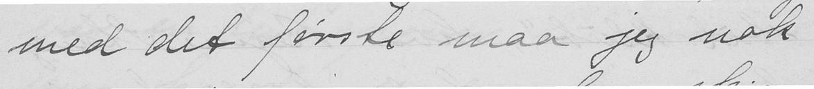med det første maa jeg nok
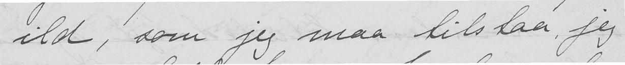ild, som jeg maa tilstaa, jeg
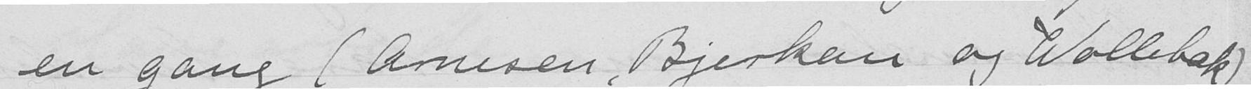en gang (Arnesen, Bjerkan og Wollebæk)
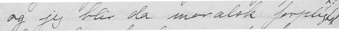og jeg blir da moralsk forpligtet
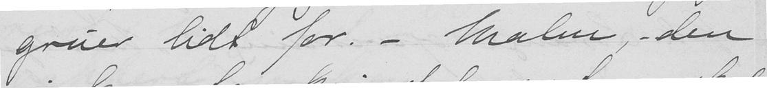gruer lidt for. - Malm, - den
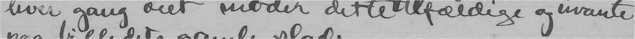hver gang øiet møder dette tilfældige og uvante
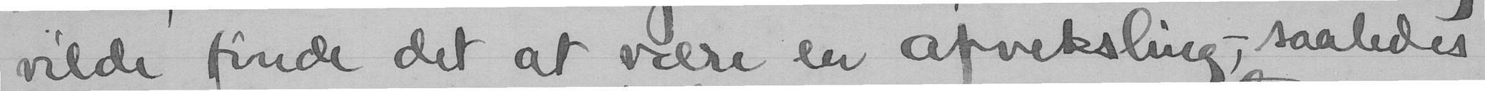vilde finde det at være en afveksling, - saaledes
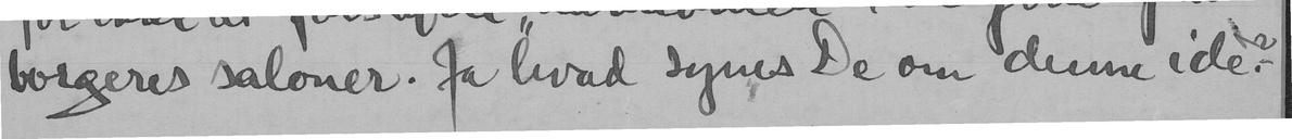borgeres saloner. Ja hvad synes De om denne ide?
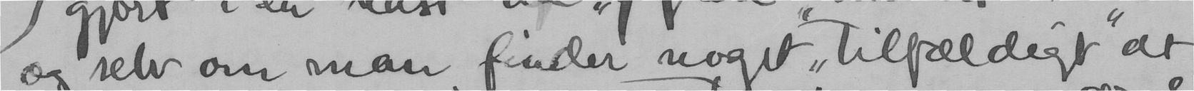og selv om man finder noget "tilfældigt" at
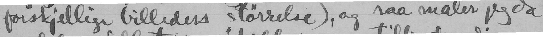forskjellige billeders størrelse), og saa maler jeg da
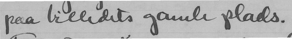paa billedets gamle plads.
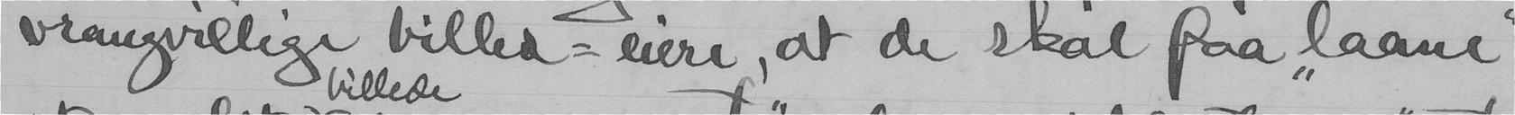vrangvillige billed-eiere, at de skal faa "laane"
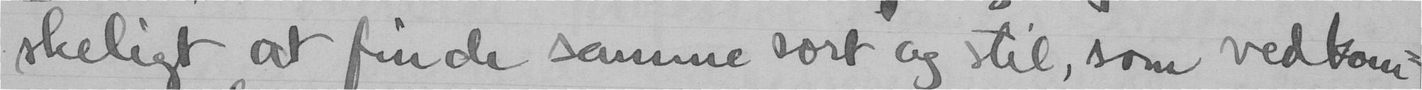skeligt at finde samme sort og stil, som vedkom-
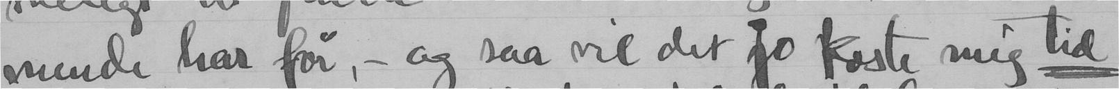mende har før, - og saa vil det jo koste mig tid
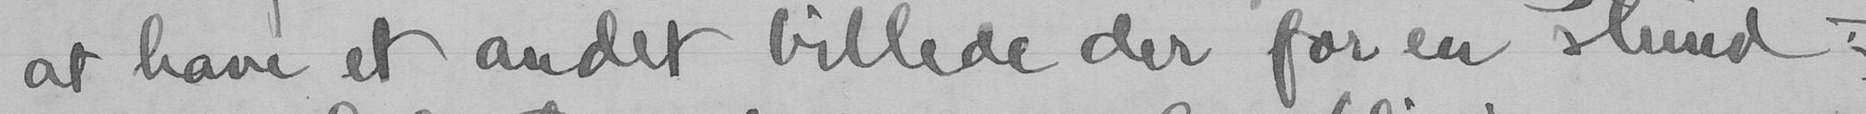at have et andet billede der for en stund -
